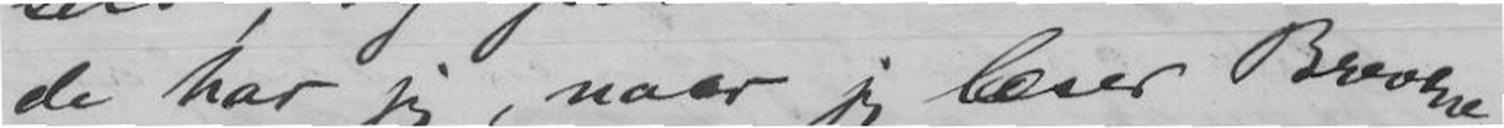de har jeg, naar jeg læser Brevene,
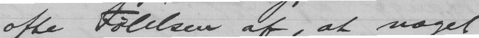ofte Følelsen af, at noget
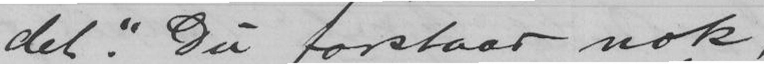det". Du forstaar nok,
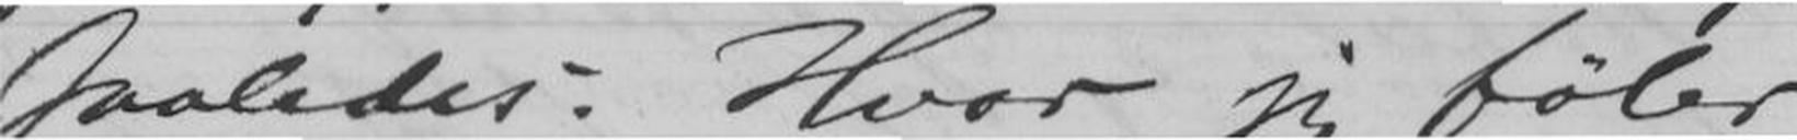saaledes. Hvor jeg føler
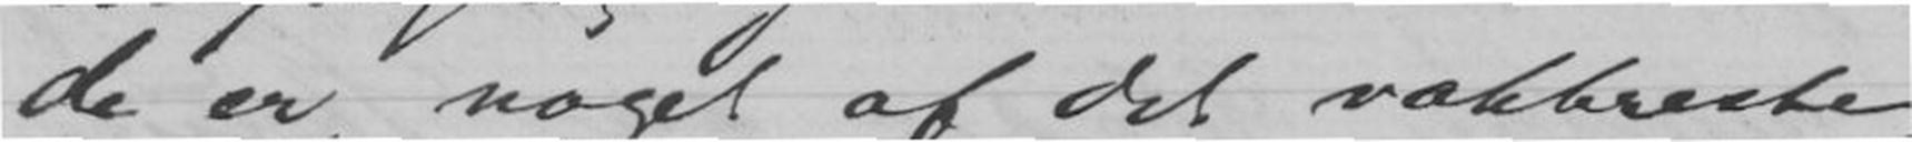de er noget af det vakkreste,
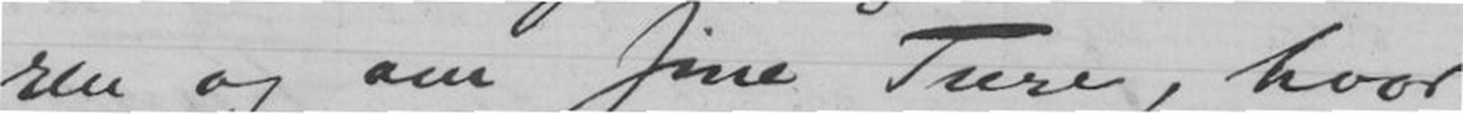ren og om sine Ture, hvor
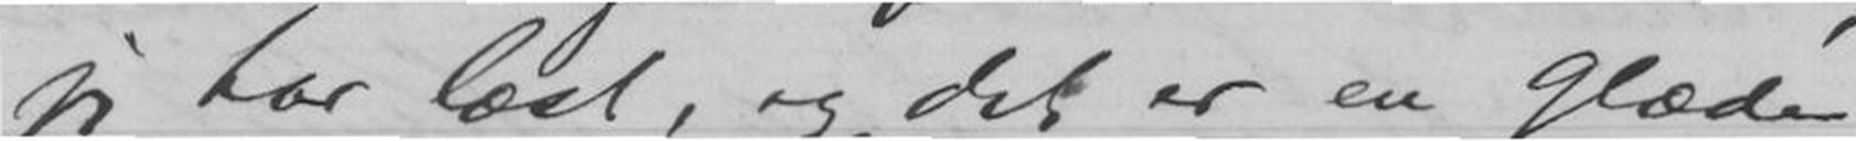jeg har læst, og det er en Glæde
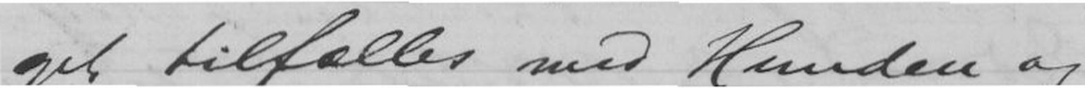get tilfælles med Hunden og
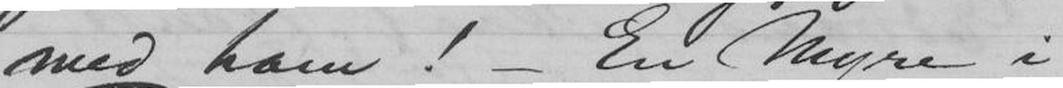med ham! - En Myre i
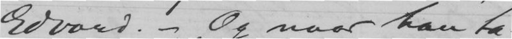Edvard. - Og naar han ta-
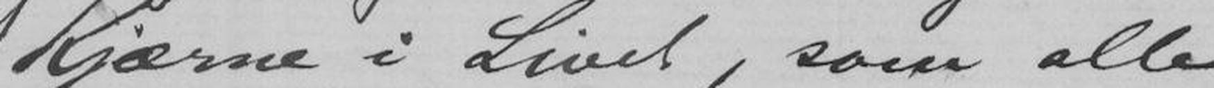Kjærne i Livet, som alle
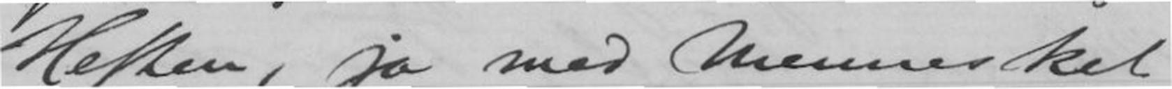Hesten, ja med Mennesket
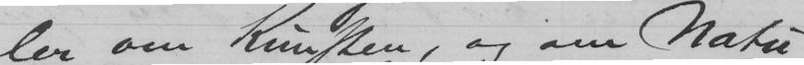ler om Kunsten, og om Natu-
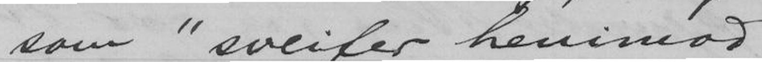som "sveifer henimod
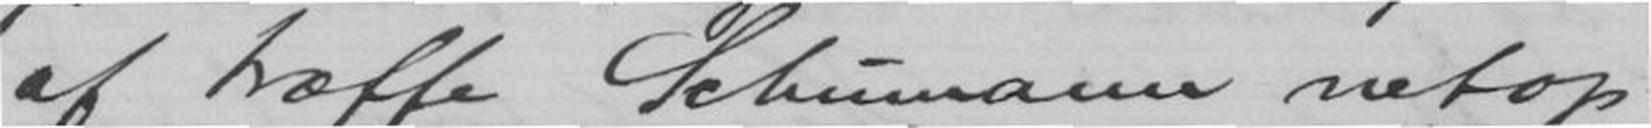at træffe Schumann netop
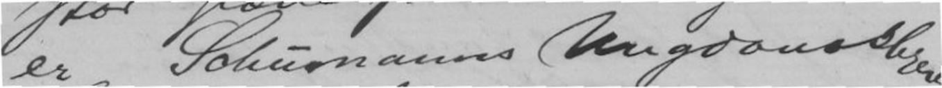er Schumanns Ungdomsbreve!
